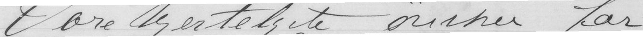Vore hjerteligste ønsker for
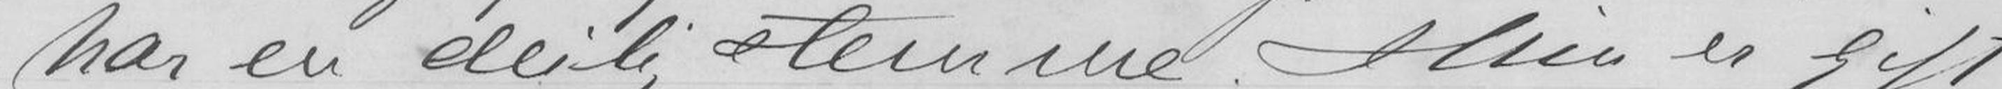har en deilig stemme. Hun er gift
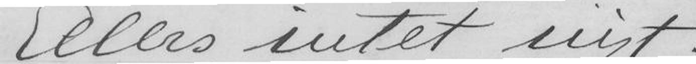Ellers intet nyt.
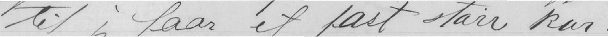til jeg faar et fast større kor.
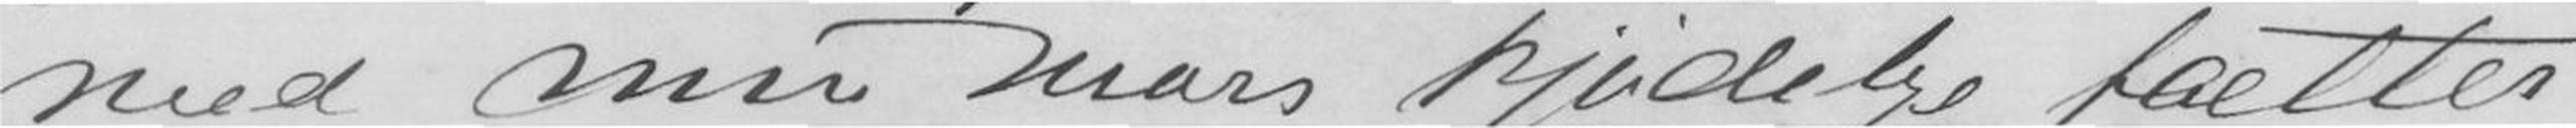med min mors kjødelige fætter.
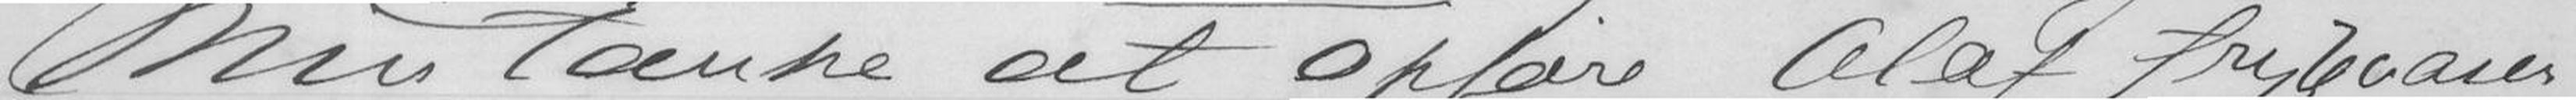Min Tanke at opføre Olaf Trygvason
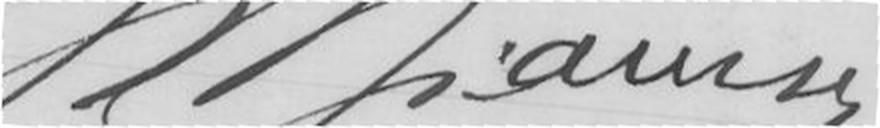B Bjørnson
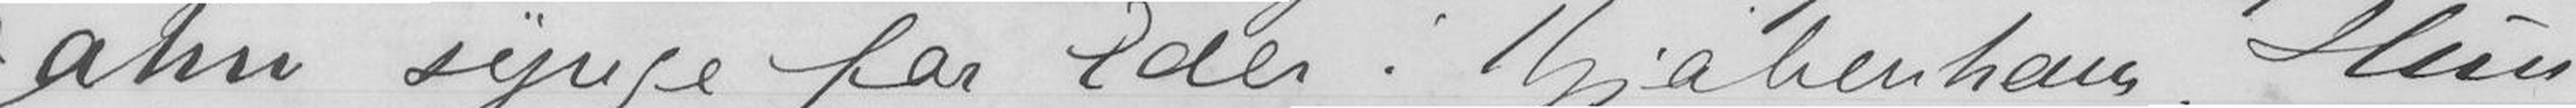Jahn synje for Eder i Kjøbenhavn. Hun
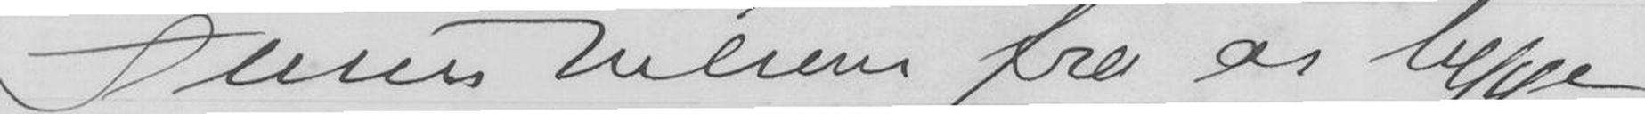Tusen hilsen fra os begge
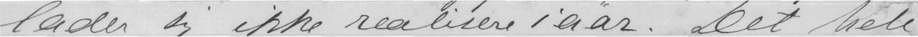lader sig ikke realisere i aar. Det hele
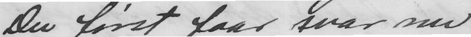Du først faar svar nu
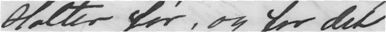Holter før, og for det
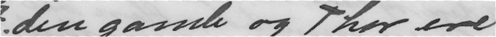den gamle og Thor ere
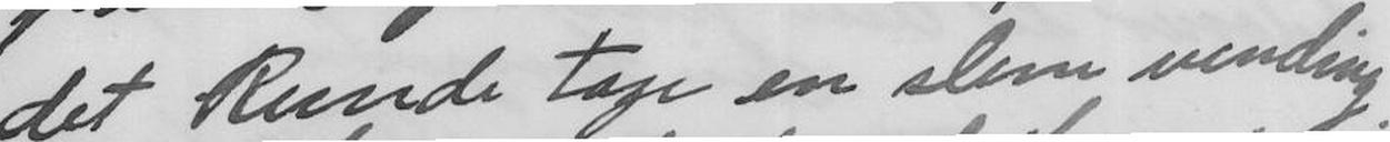det kunde tage en slem vending.
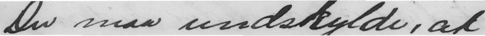Du maa undskylde, at
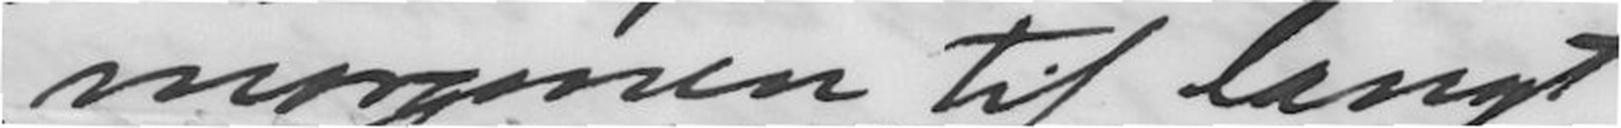morgenen til langt
